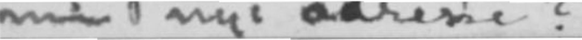min nye adresse?
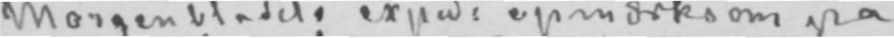Morgenbladets exped: opmærksom på
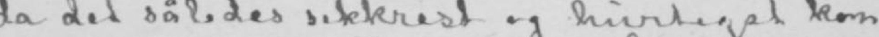da det således sikkrest og hurtigst kom-
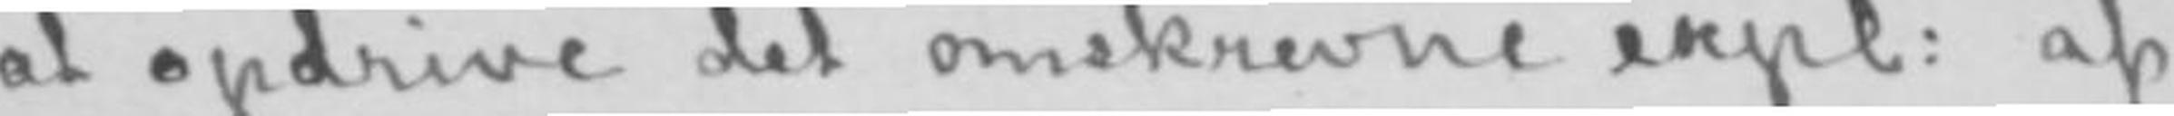at opdrive det omskrevne expl: af
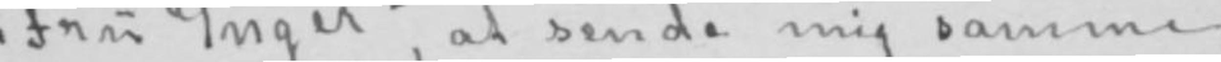«Fru Inger», at sende mig samme
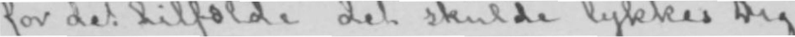for det tilfælde det skulde lykkes Dig
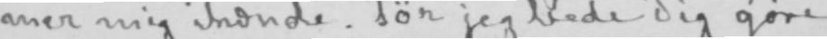mer mig ihænde. Tør jeg bede Dig gøre
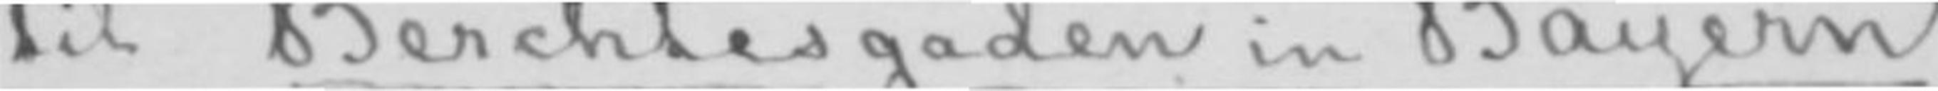til Berchtesgaden in Bayern,
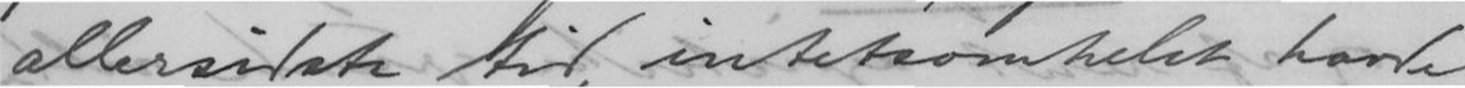allersidste tid, intetsomhelst havde
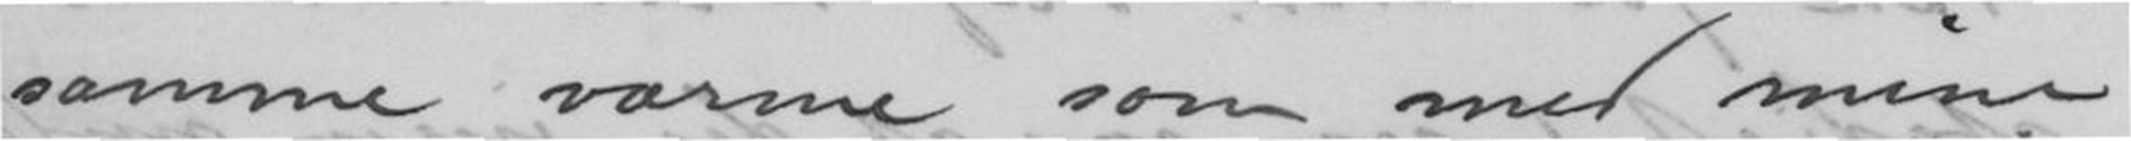samme varme som med mine
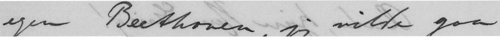egen Beethoven, jeg vilde gaa
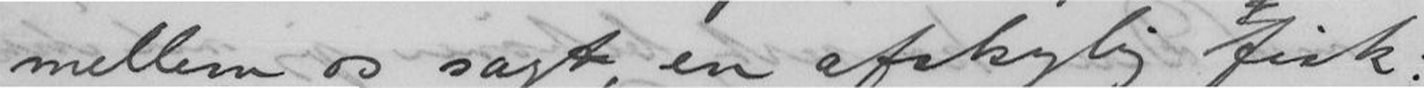mellem os sagt, en afskylig fisk! -
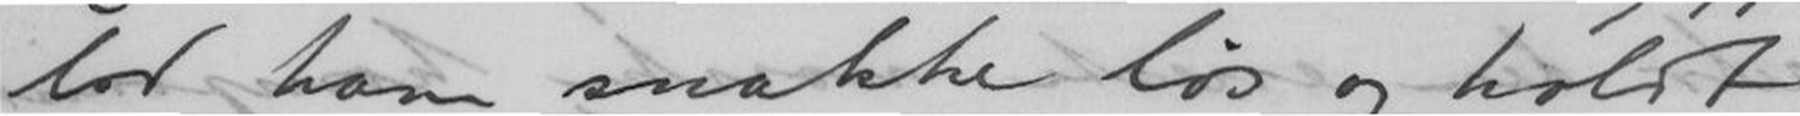lod ham snakke løs og holdt
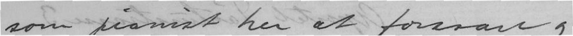som pianist her at forsvare og
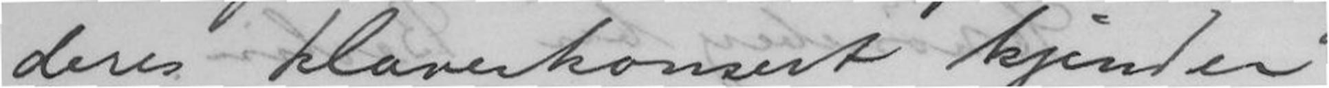deres klaverkonsert kjender
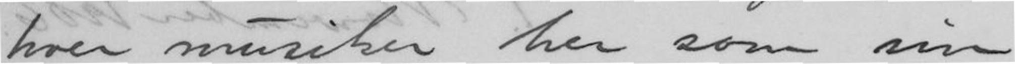hver musiker her som sin
